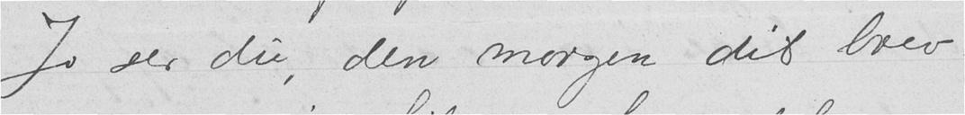Jo ser du, den morgen dit brev
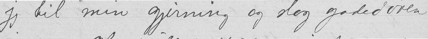jeg til min gjerning og slag gadedøren
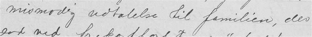mismodig udtalelse til familien, der
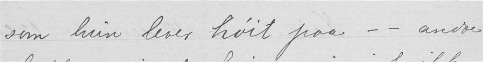som hun lever høit paa - - andre
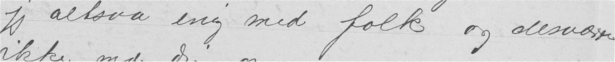jeg altsaa enig med folk og desværre
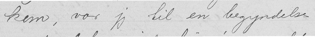kom, var jeg til en begyndelse
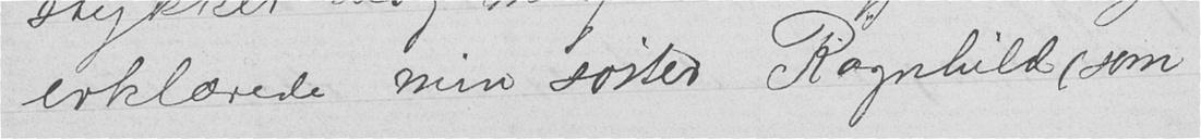erklærede min søster Ragnhild (som
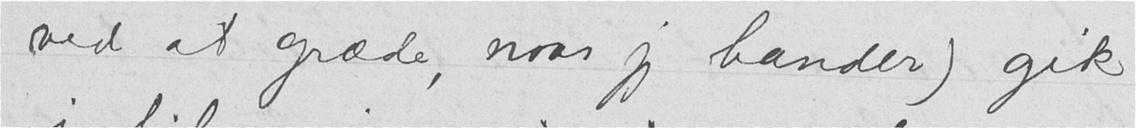ved at græde, naar jeg bander) gik
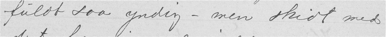fuldt saa yndig - men skidt med
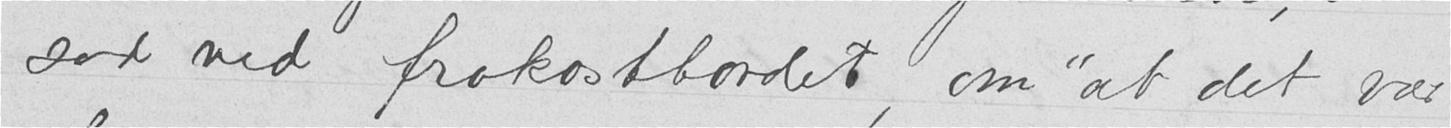sad ved frokostbordet, om "at det var
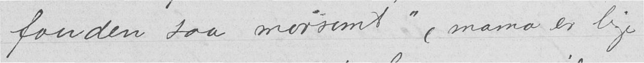fanden saa morsomt" (mama er lige
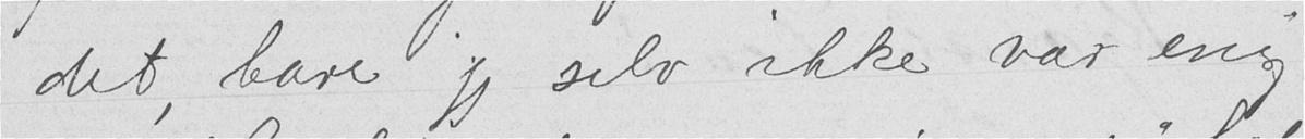det, bare jeg selv ikke var enig
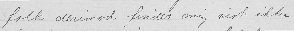folk derimod finder mig vist ikke
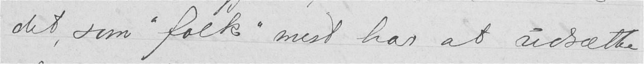dit, som "folk" mest har at udsætte
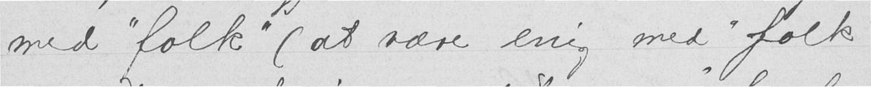med "folk" (at være enig med "folk"
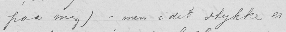paa mig) - men i det stykke er
